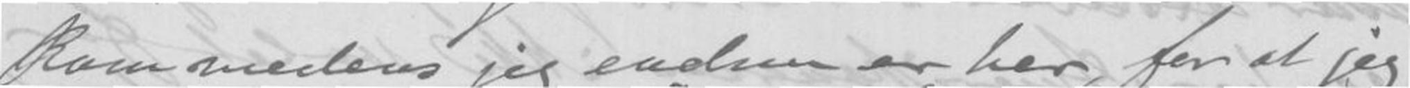kom medens jeg endnu er her, for at jeg
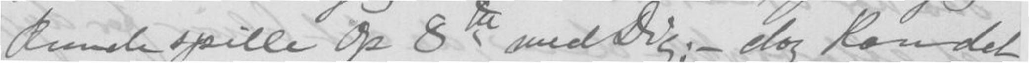kunde spille Op 8th med Dig; - dog kan det
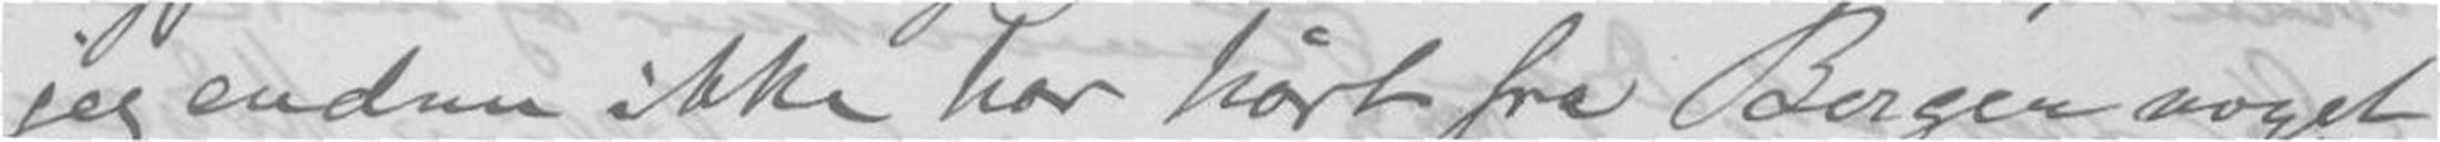jeg endnu ikke har hørt fra Bergen noget
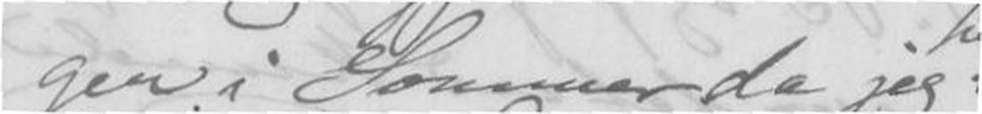gen i Sommer da jeg
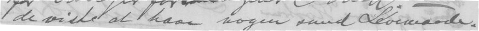de vistge at have nogen sand Levemaade.
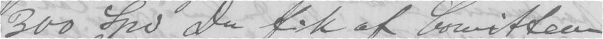300 Spd Du fik af Comitteen
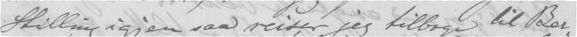Stilling igjen saa reiser jeg tilbage til Ber-
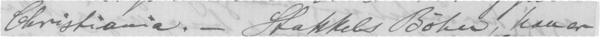Christiania. - Stakkels Bøker, han er
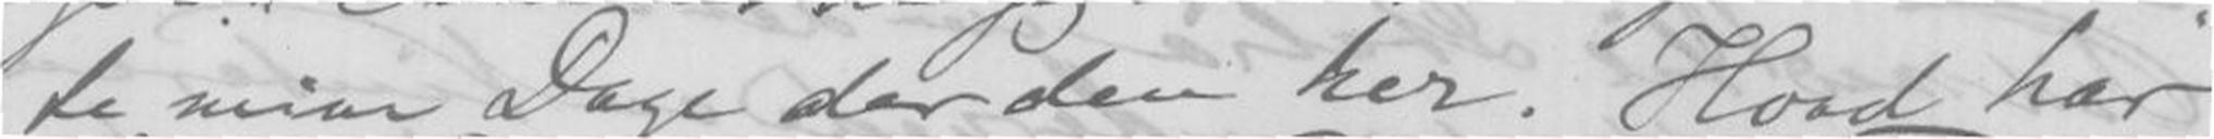te mine Dage der den her. Hvad har
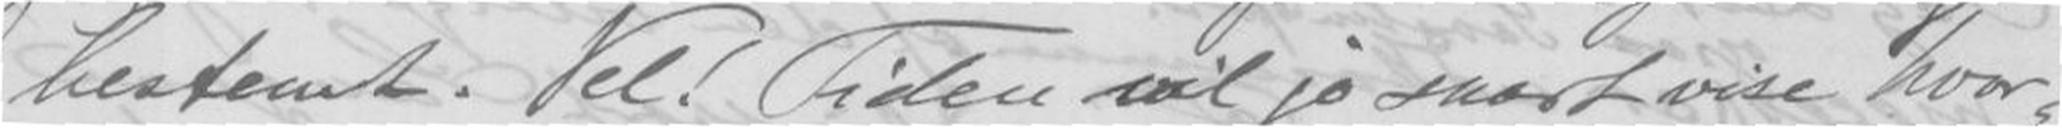bestemt. Vel! Tiden vil jo snart vise hvor-
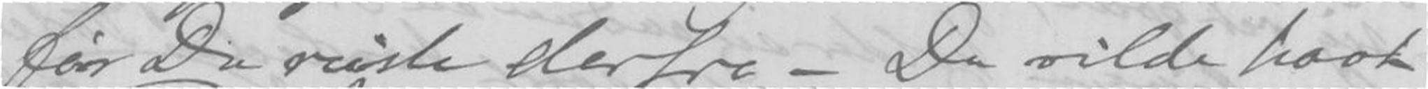før Du reiste derfra - Du vilde havt
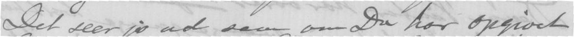Det seer jo ud som om Du har opgivet
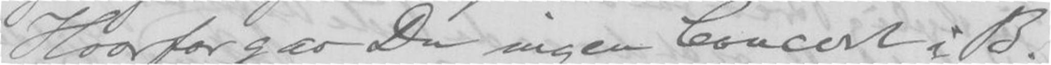Hvorfor gav Du ingen Concert i B.
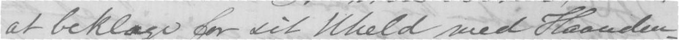at beklage for sit Uheld med Haanden
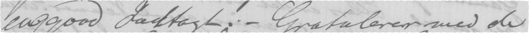en good Indtægt. - Gratulerer med de
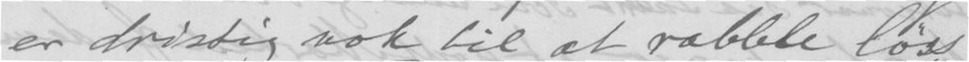er dristig nok til at rabble løss
